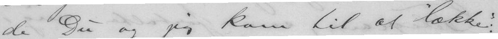de Du og jeg kom til at "lække":
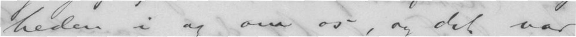heden i og om os, og det var
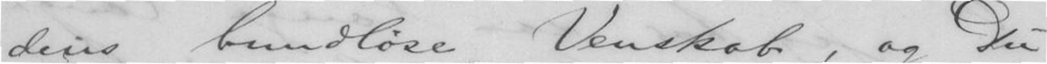dens bundløse Venskab, og Du
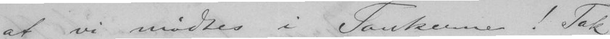at vi mødtes i Tankerne! Tak
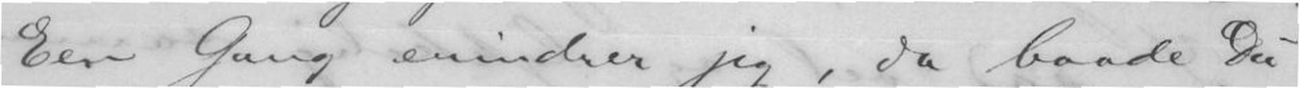Een Gang erindrer jeg, da baade Du
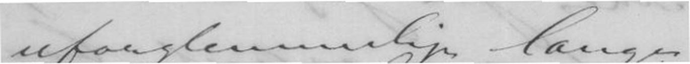uforglemmelige lange
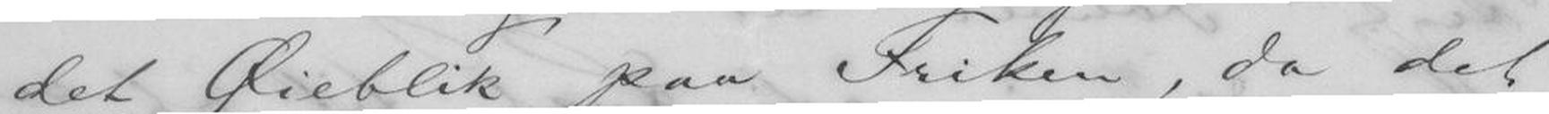det Øieblik paa Friken, da det
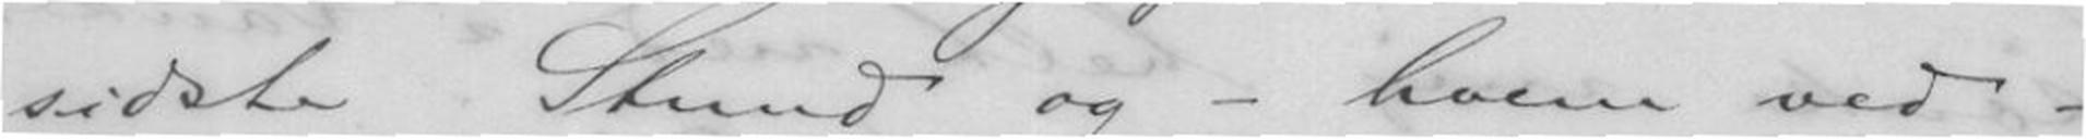sidste Stund og - hvem ved -
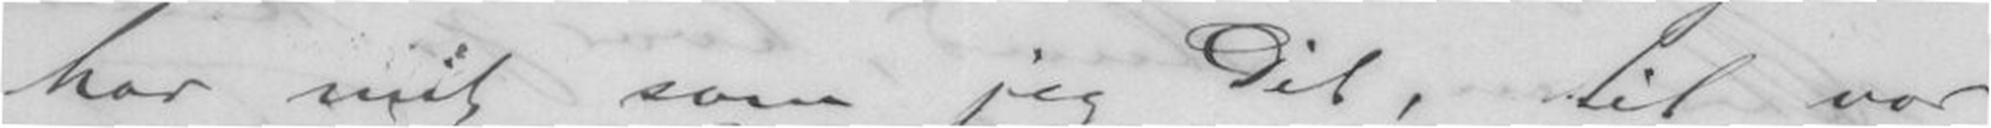har mit som Dit, til vor
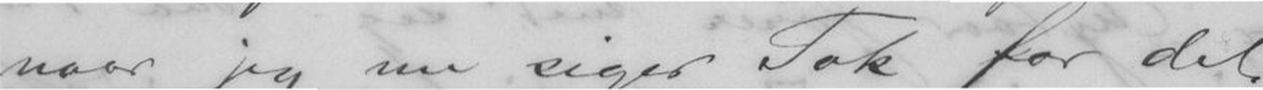naar jeg siger Tak for det
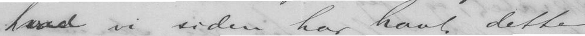hvad vi siden har havt dette
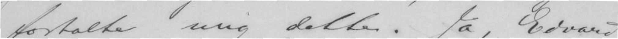fortalte mig dette. Ja, Edvard,
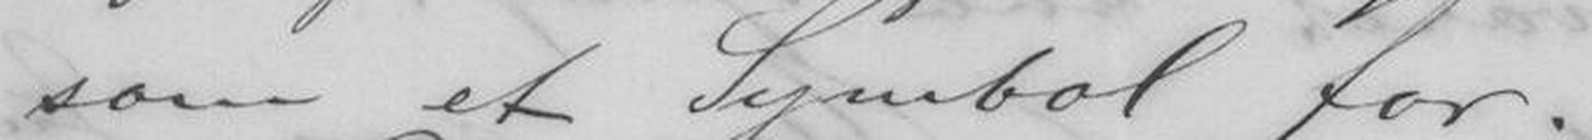som et Symbol for.
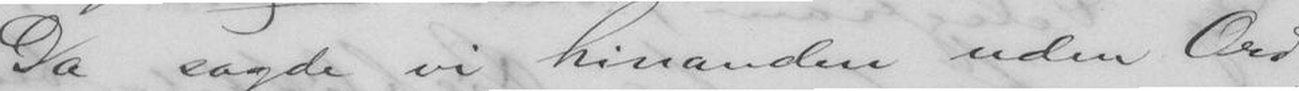Da sagde vi hinanden uden Ord,
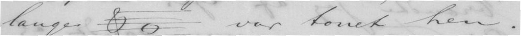lange noter: g var tonet hen.
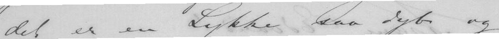det er en Lykke saa dyb og
